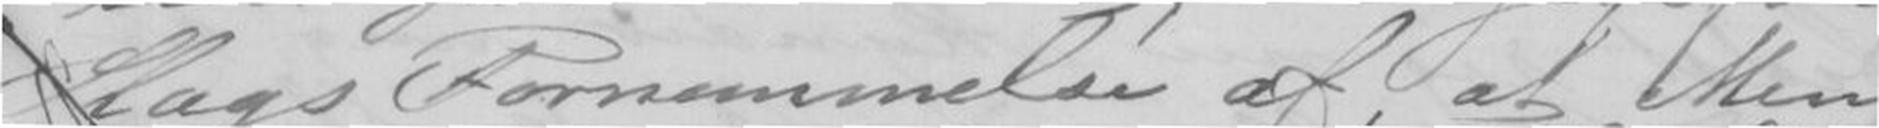Slags Fornemmelse af, at Men
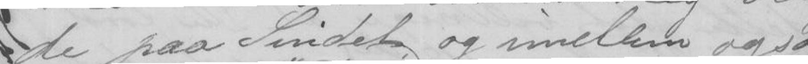de paa Sindet, og imellem ogsa
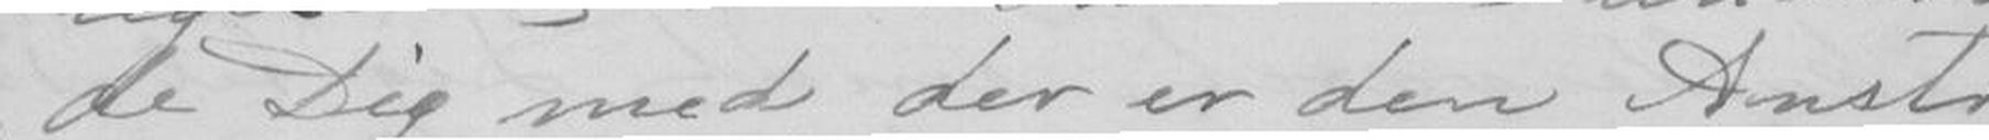de Dig med, der er den Anst
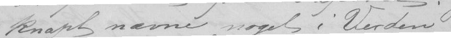knapt nævne noget i Verden,
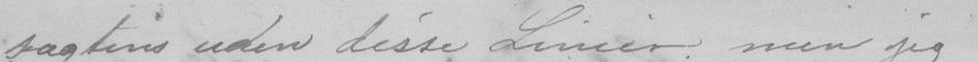sagtens uden disse Linier; men jeg slutter
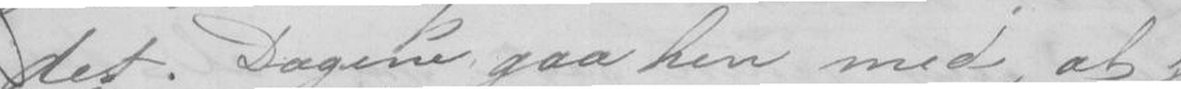det. Dagene gaa hen med, at
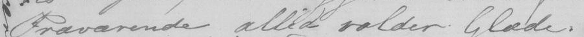Fraværende, altid volder Glæde. -
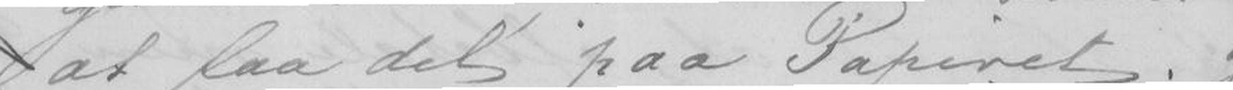at faa det pa Papiret.
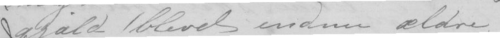gjæld blevet endnu ældre
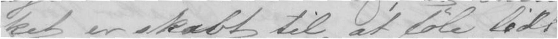ket er skabt til at føle lidt
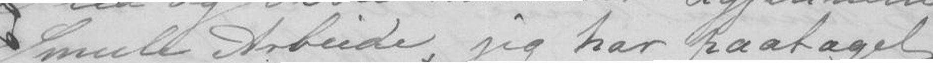Smule Arbeide jeg har paataget
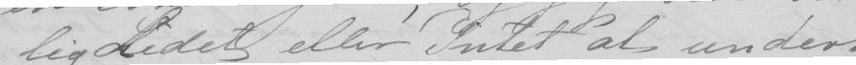lig Lidet eller Intet at under
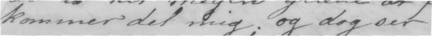kommer det mig; og dog ser
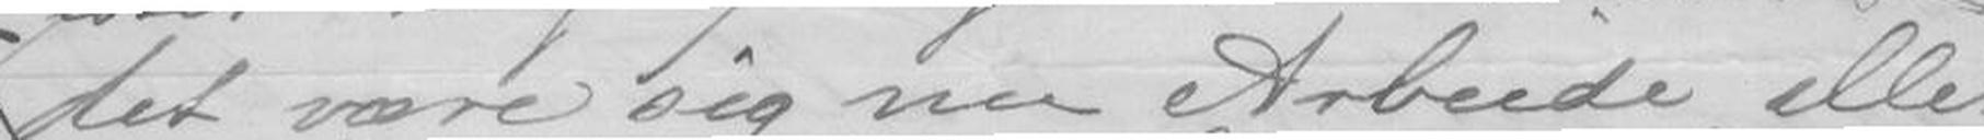det være sig nu Arbeide elle
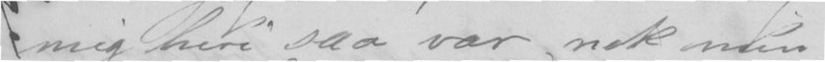mig heri saa var nok min Brev-
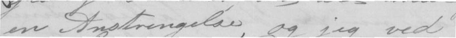en Anstrengelse, og jeg ved virke-
